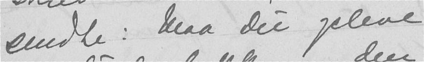sendte: Maa du opleve
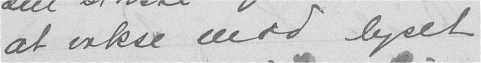at vokse mod lyset
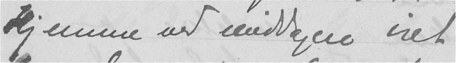Hjemme ved middagen viet
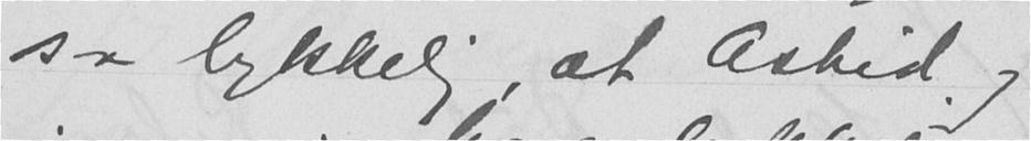saa lykkelig, at Astrid og
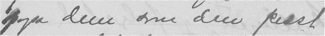papa dem som den præst
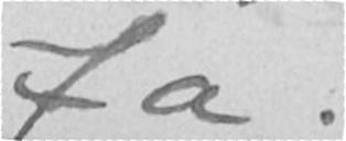Ja.
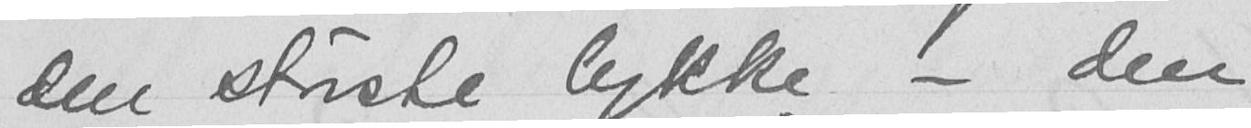den største lykke - den
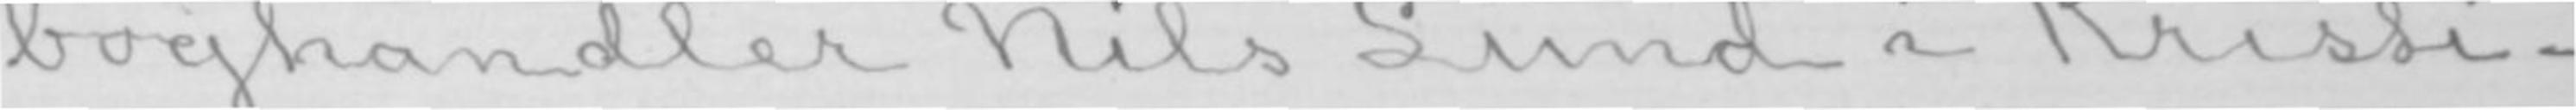boghandler Nils Lund i Kristi-
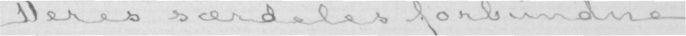Deres særdeles forbundne
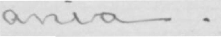ania.
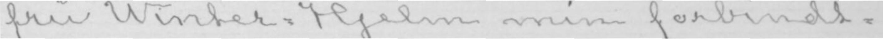fru Winter-Hjelm min forbindt-
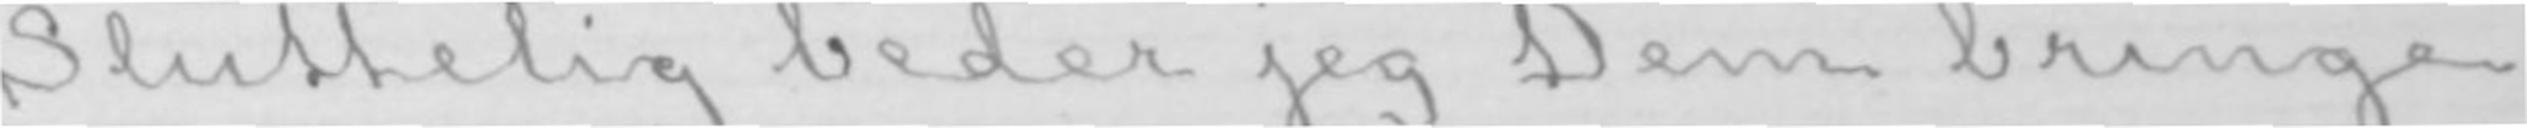Sluttelig beder jeg Dem bringe
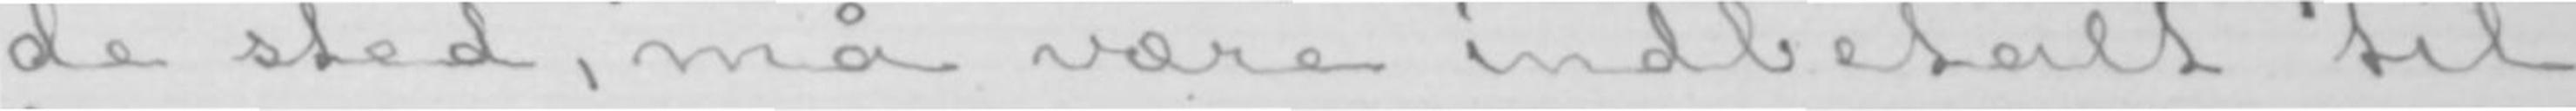de sted, må være indbetalt til
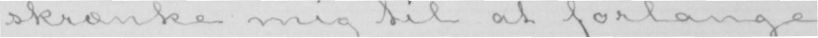skrænke mig til at forlange
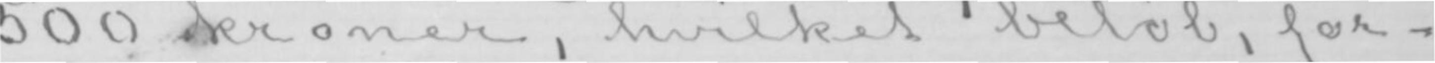500 kroner, hvilket beløb, for-
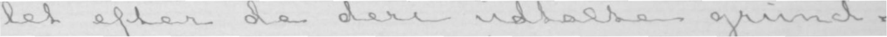let efter de deri udtalte grund-
